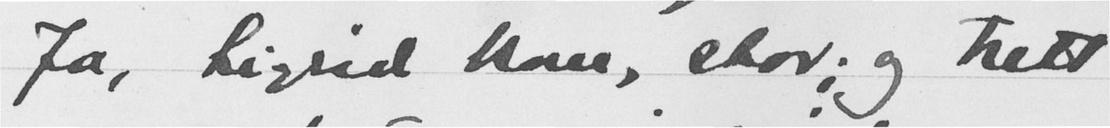Ja, Sigrid kone, stor; og trett
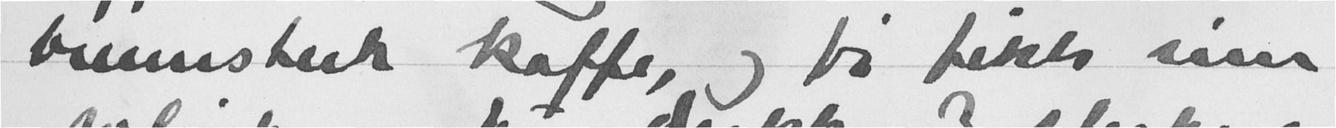brennsterk kaffe, og vi fikk inn
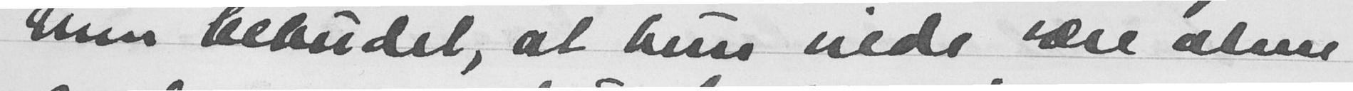hun bebudet, at hun vilde være alene
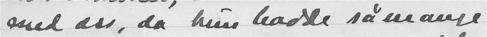med oss, da hun hadde såmange
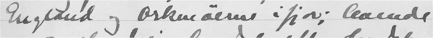England og Orkenøiene ifjor; levende
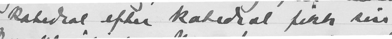katedral efter katedral fikk sin
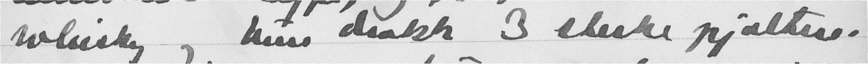whisky og hun drakk 3 sterke pjaltere.
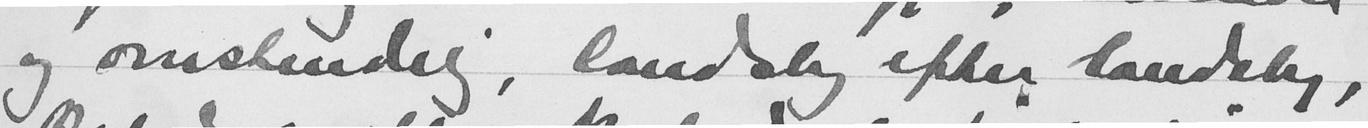og omstendelig, landsby efter landsby,
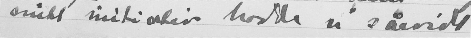mitt initiativ hadde vi såvidt
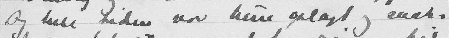Og hele tiden var hun oplagt og snak-
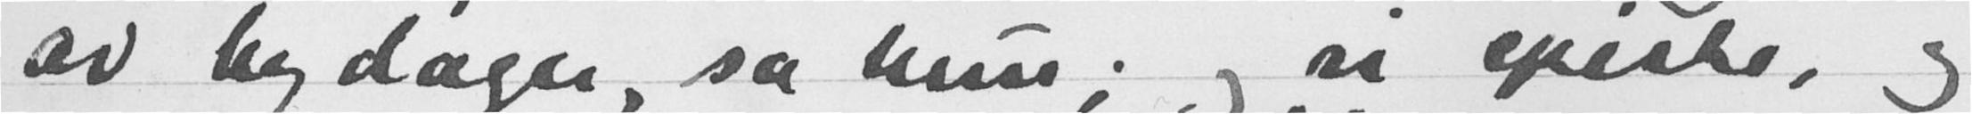av bydager, sa hun; og vi spiste, og
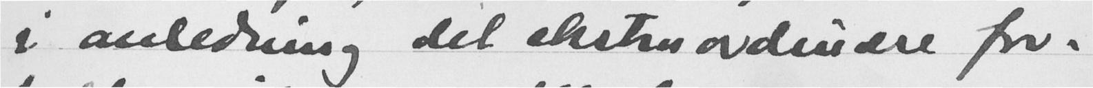i anledning det ekstraordinære for-
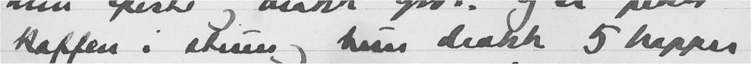kaffen i stuen og hun drakk 5 kopper
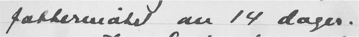fattermøtet om 14 dager.
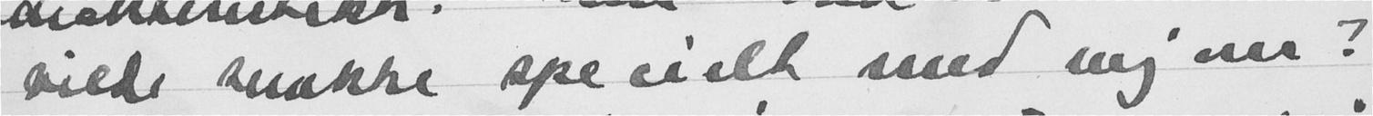vilde snakke specielt med mig om?
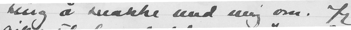ting å snakke med mig om. Jeg
